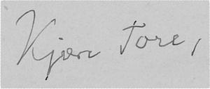Kjære Tore,
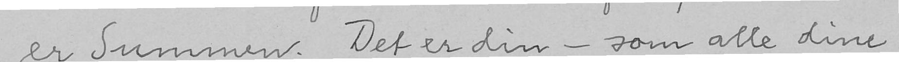er Summen. Det er din - som alle dine
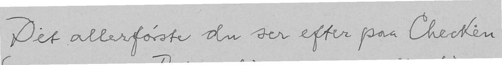Det allerførste du ser efter paa Checken
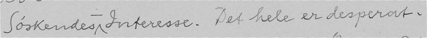Søskendes - Interesse. Det hele er desperat.
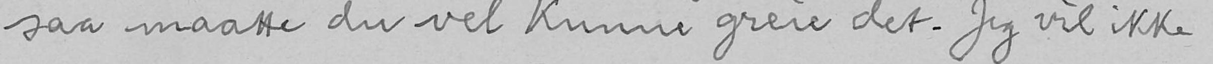saa maatte du vel kunne greie det. Jeg vil ikke
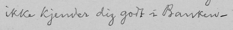ikke kjender dig godt i Banken.
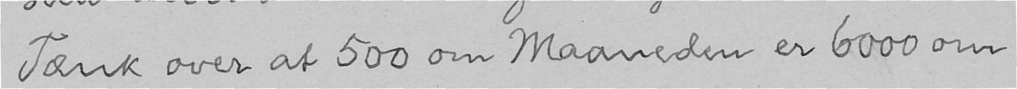Tænk over at 500 om Maaneden er 6000 om
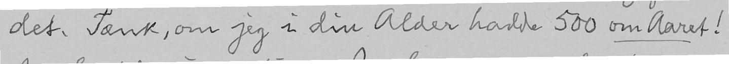det. Tænk, om jeg i din Alder hadde 500 om Aaret!
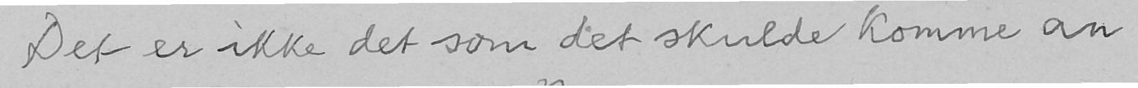Det er ikke det som det skulde komme an
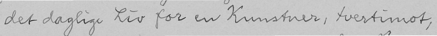det daglige Liv for en Kunstner, tvertimot,
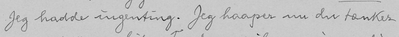Jeg hadde ingenting. Jeg haaper nu du tænker
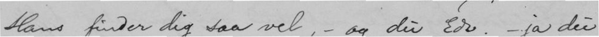Hans finder dig saa vel, - og du Edv. - ja du
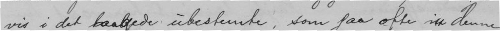vis i det baagede ubestemte, som saa ofte i denne
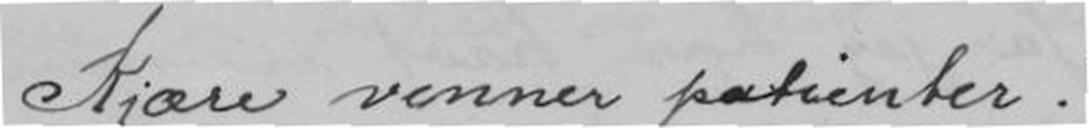Kjære venner patienter!
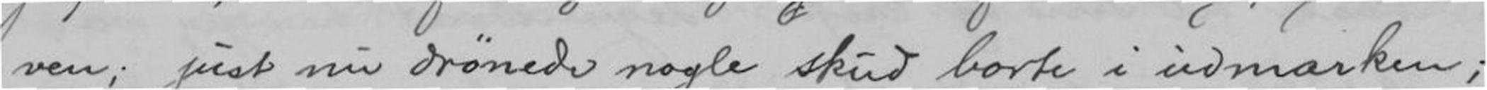ven, just nu drønede nogle skud borte i udmarken;
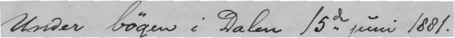Under bøgen i Dalen 15de juni 1881.
